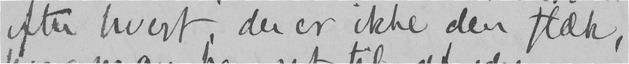efter hvert, der er ikke den flæk,
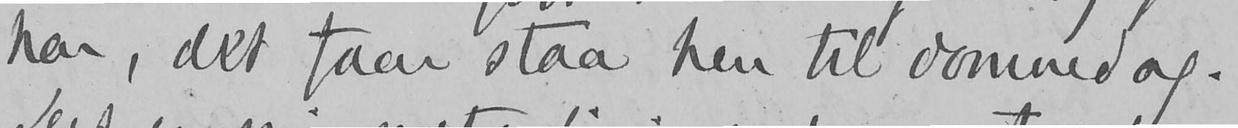har, det faar staa hen til dommedag.
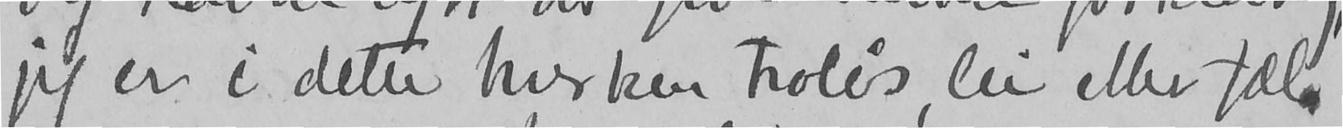jeg er i dette hverken troløs, lei eller fæl.
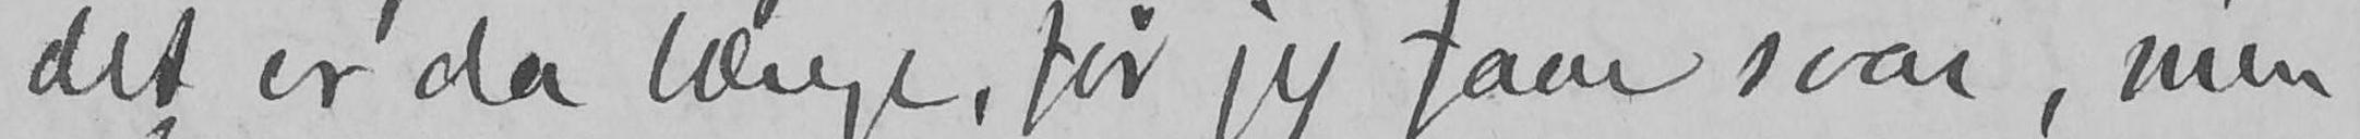det er da længe, før jeg faar svar, men
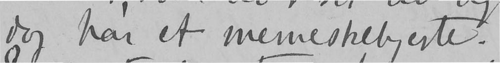dog har et menneskehjerte.
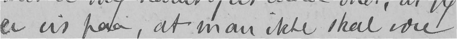er vis paa, at man ikke skal være
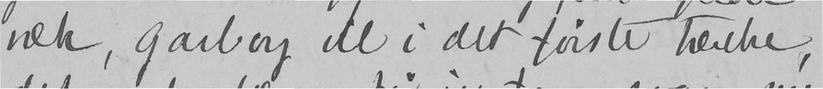væk, Garborg vil i det første tænke,
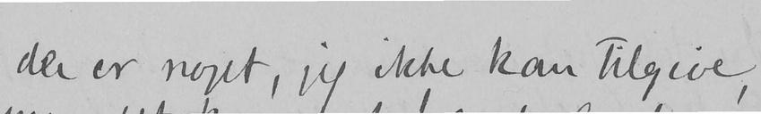der er noget, jeg ikke kan tilgive,
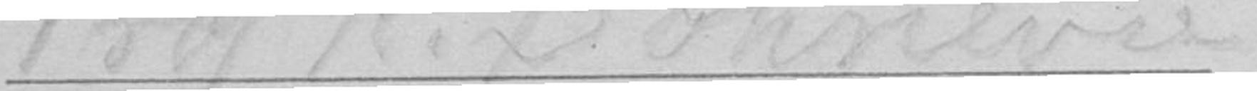Prof. K. Bonnevie
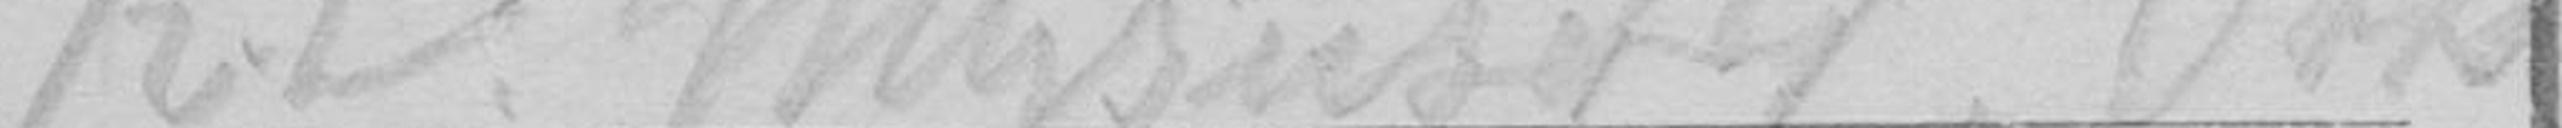p.t. Mysuseter, Otta
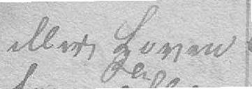eller Loven
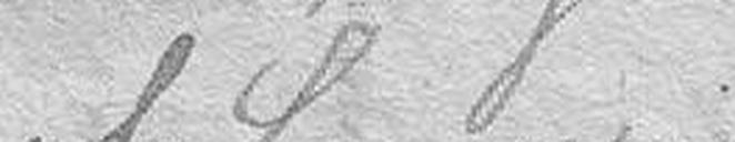naar noget
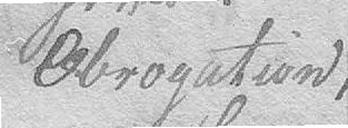Abrogation
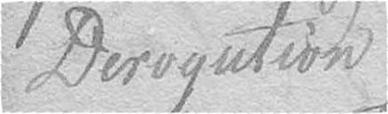Derogation
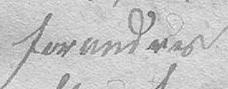forandres
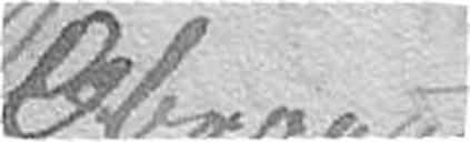hæves.
